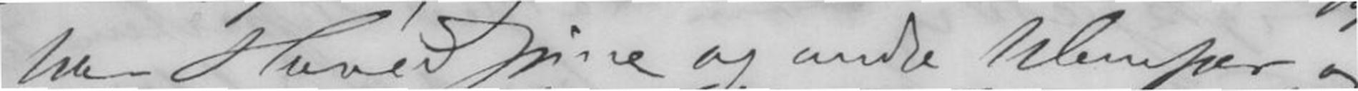har Hovedpine og andre Ulemper og
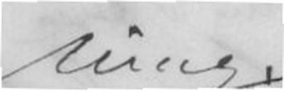ning.
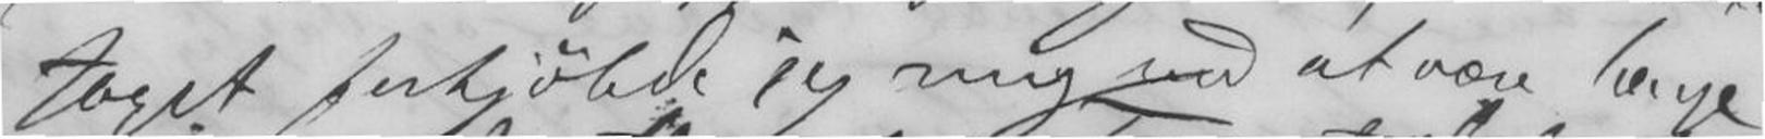toget forkjølede jeg mig ved at være længe
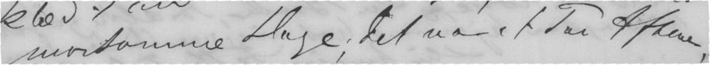morsomme Dage; det var et Par Aftene,
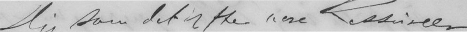Dig som det efter vore Ressurcer
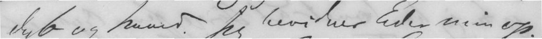dyb og haard. Jeg bevidner Eder min op-
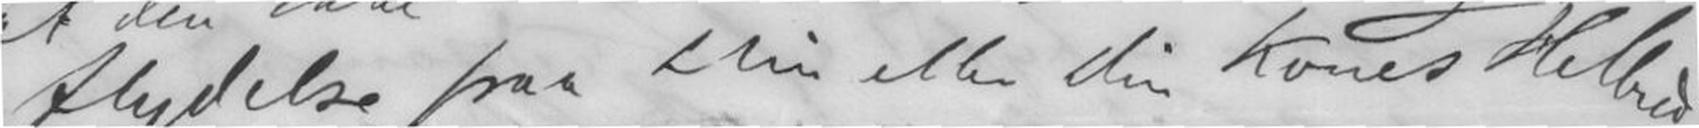flydelse paa Dig eller Din Kones Helbred.
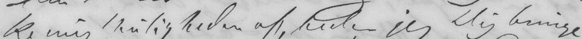ke mig Muligheden af, bede jeg Dig bringe
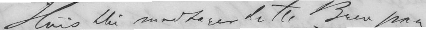Hvis Du modtager dette Brev paa
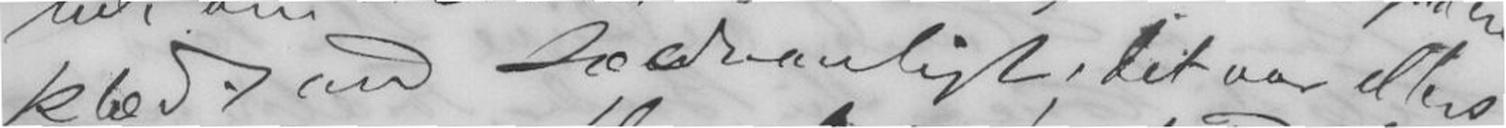klædt end sædvanligt; det var ellers
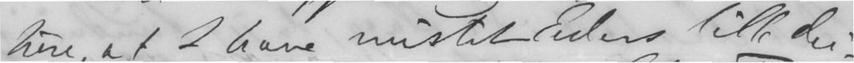høre, at I have mistet Eders lille Dei-
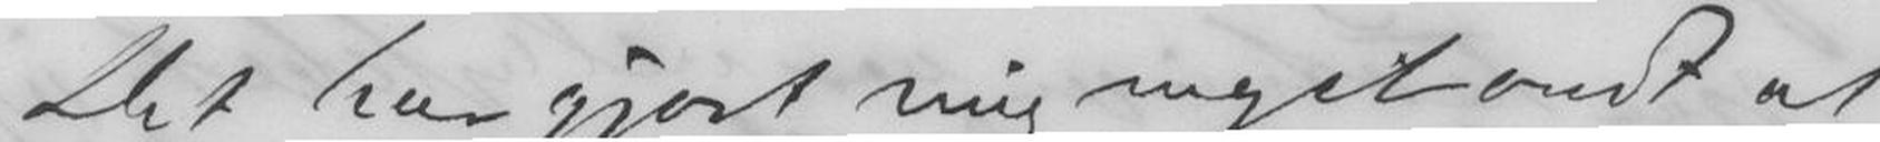Det har gjort mig meget ondt at
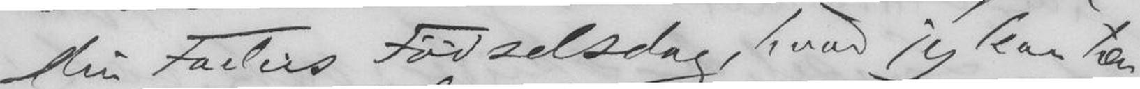Din Faders Fødselsdag, hvad jeg kan tæn
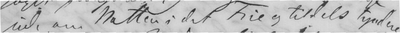ude om Natten i det Frie og tildels tyndere
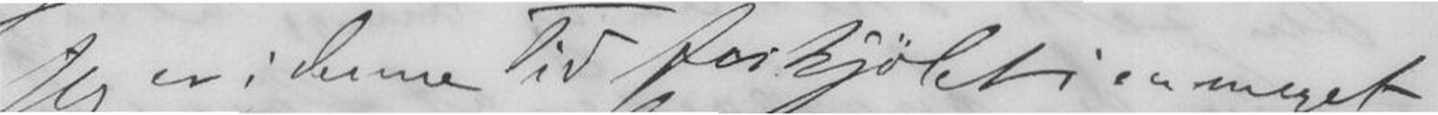Jeg er i denne Tid forkjølet i en meget
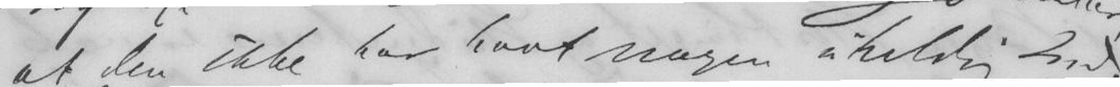at den ikke har havt nogen uheldig Ind
